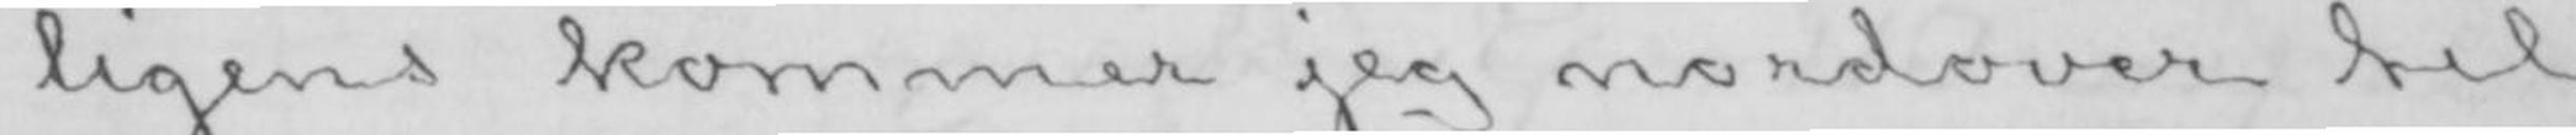ligens kommer jeg nordover til
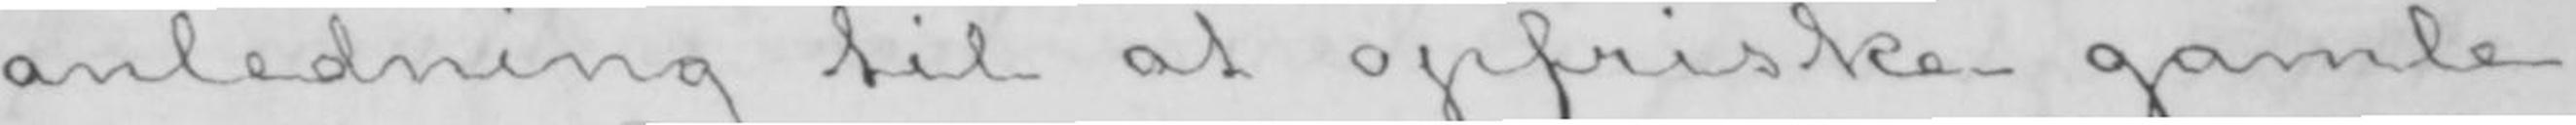anledning til at opfriske gamle
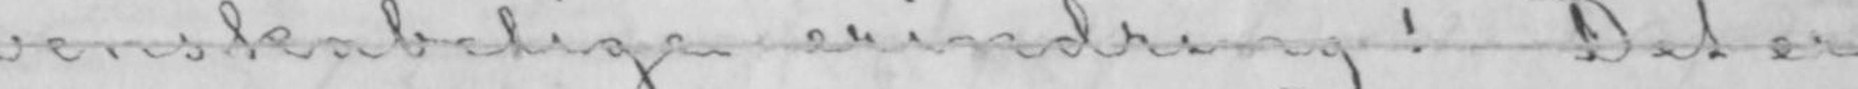venskabelige erindring! Det er
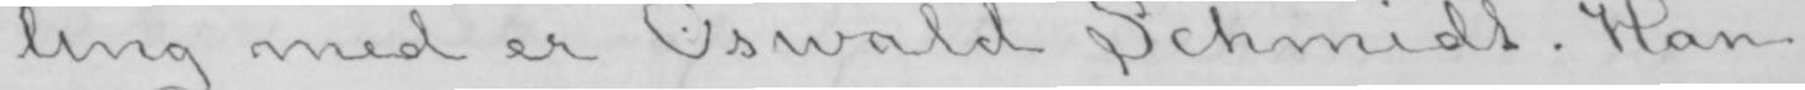ling med er Oswald Schmidt. Han
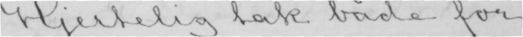Hjertelig tak både for
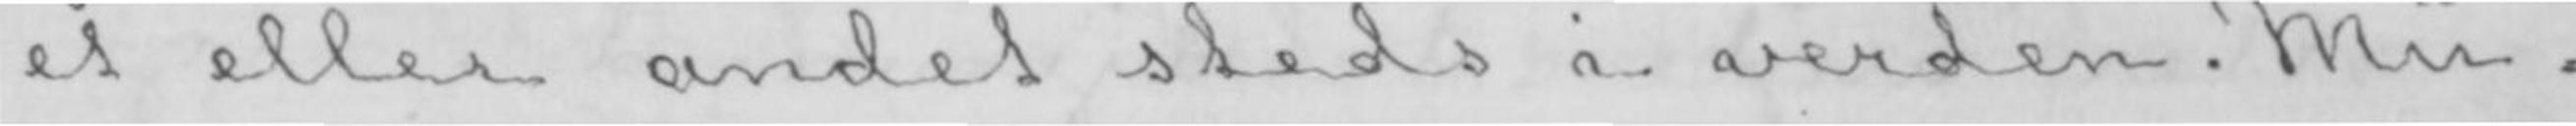et eller andet steds i verden. Mu-
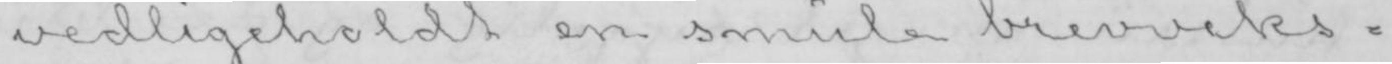vedligeholdt en smule brevveks-
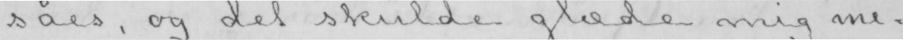såes, og det skulde glæde mig me-
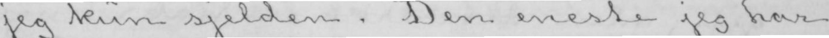jeg kun sjelden. Den eneste jeg har
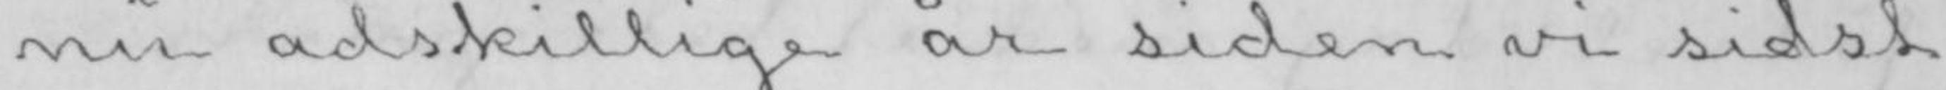nu adskillige år siden vi sidst
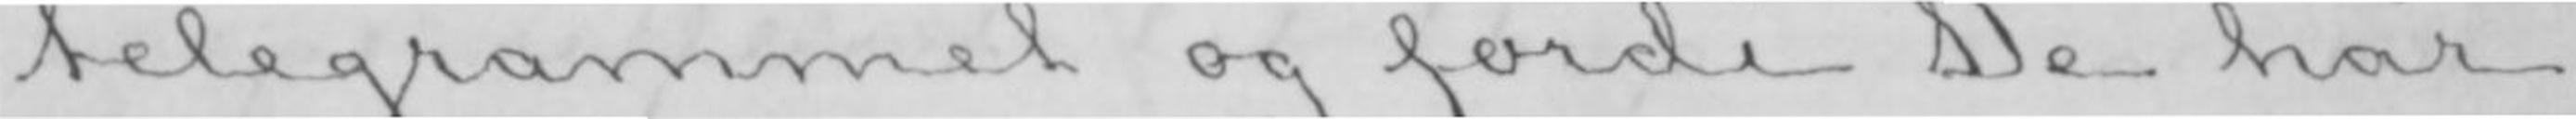telegrammet og fordi De har
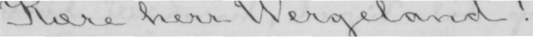Kære herr Wergeland!
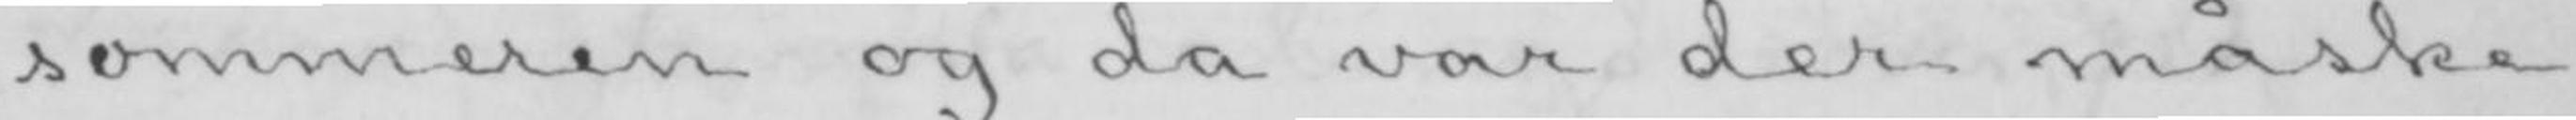sommeren og da var der måske
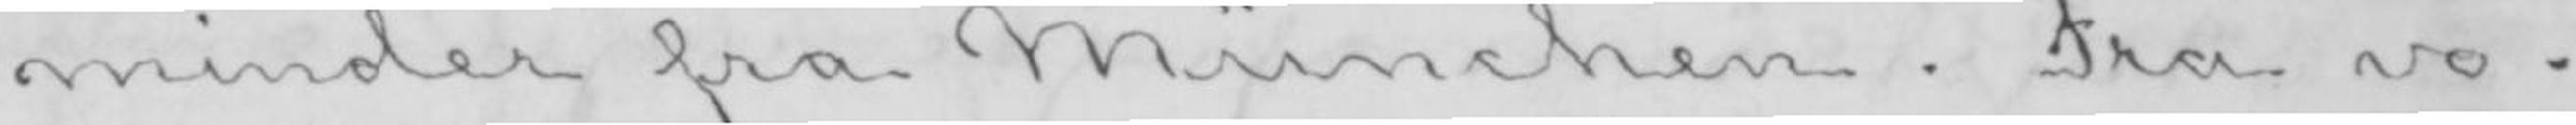minder fra München. Fra vo-
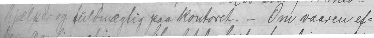hjælper og fuldmægtig paa kontoret. - Om vaaren ef-
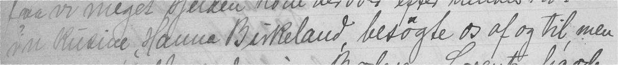min kusine, Hanna Birkeland, besøgte os af og til, men
